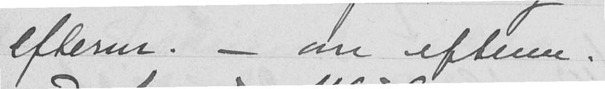efterm. - om efterm.
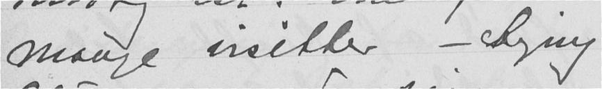mange visitter - Signy
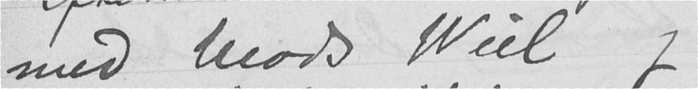med Mads Wiel og
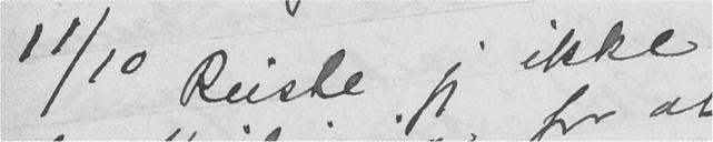11/10 Reiste jeg ikke
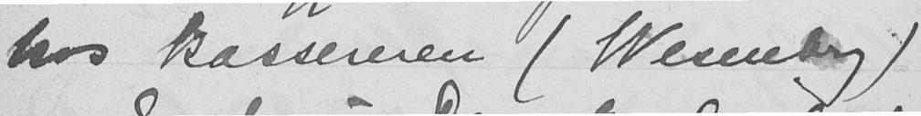hos kassereren (Wesenberg)
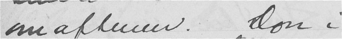om aftenen. Don i
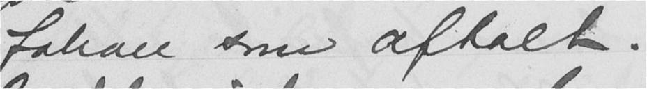Johan som aftalt.
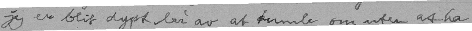jeg er blit dypt lei av at tumle om uten at ha
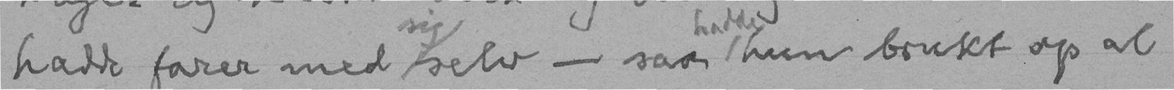hadde farer med selv - saa hun brukt op al
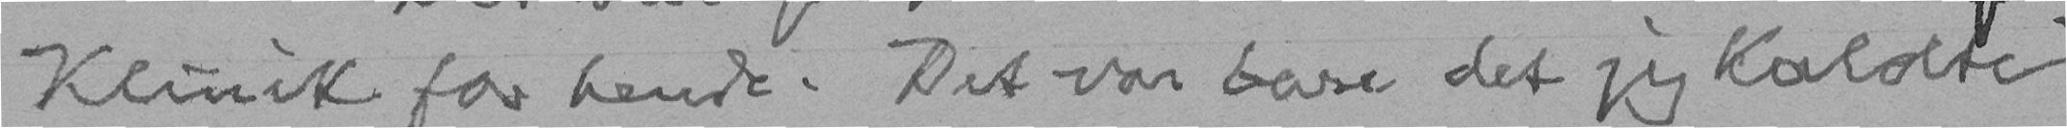Klinik for hende. Det var bare det jeg kaldte
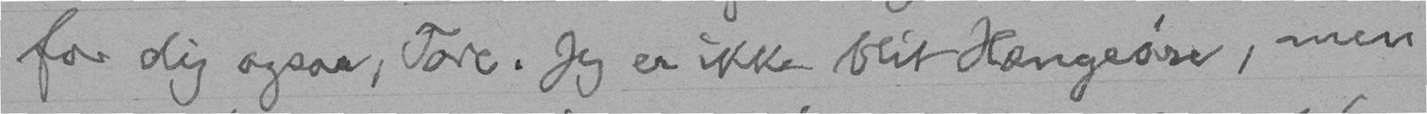for dig ogsaa, Tore. Jeg er ikke blit Hængeøre, men
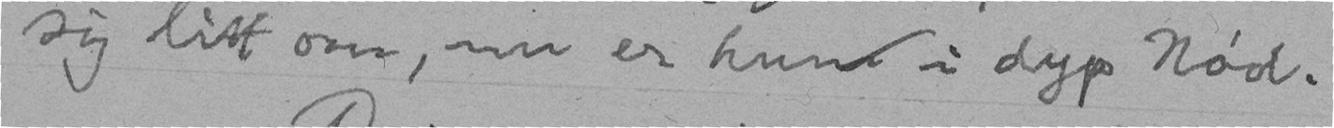sig litt om, nu er hun i dyp Nød.
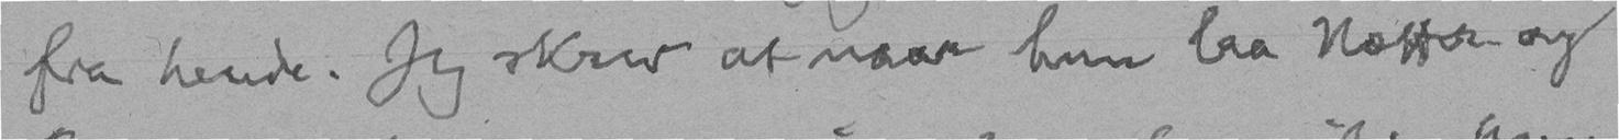fra hende. Jeg skrev at naar hun laa Nætter og
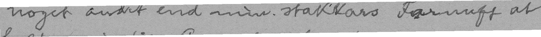noget andet end min stakkars Fornuft at
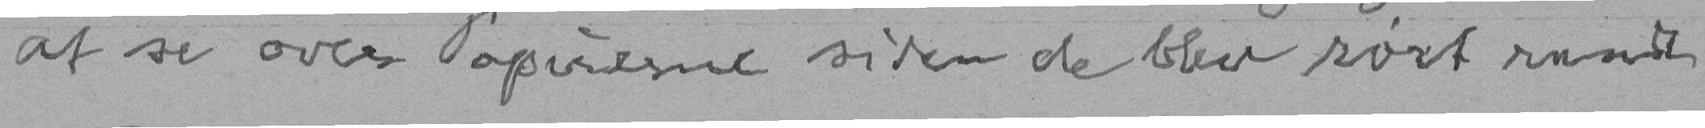at se over Papirerne siden de blev rørt rundt
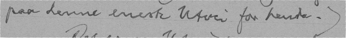paa denne eneste Utvei for hende.)
